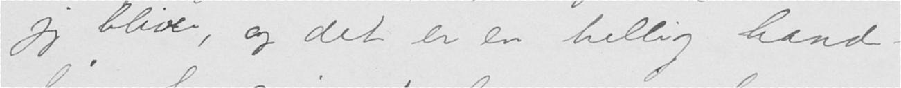jeg blive, og det er en hellig hand-
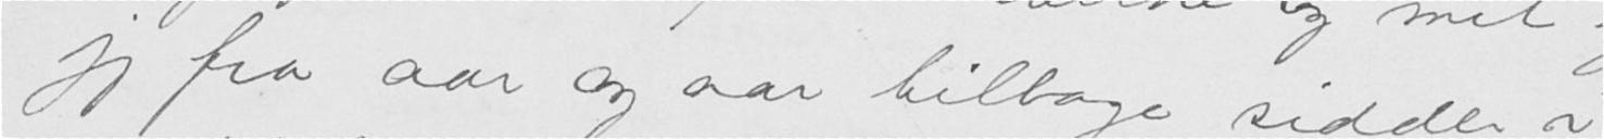jeg fra aar og aar tilbage sidder i
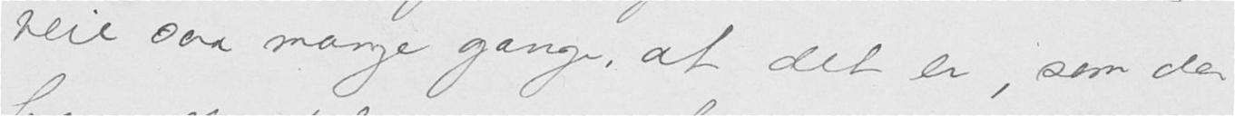veie saa mange gange, at det er, som der
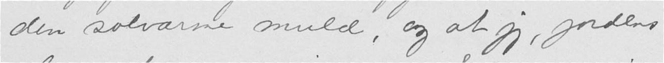den solvarme muld, og at jeg, jordens
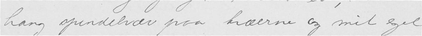hang spindelvæv paa træerne og mit eget
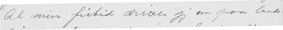Al min fritid driver jeg om paa lande-
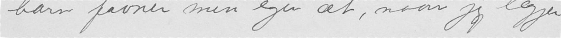barn favner min egen æt, naar jeg lægger
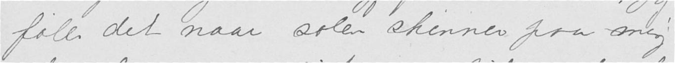føler det naar solen skinner paa mig
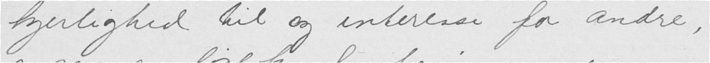kjærlighed til og interesse for andre,
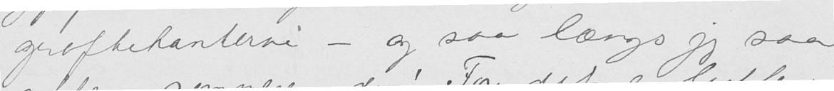grøftekanterne - og saa længes jeg saa
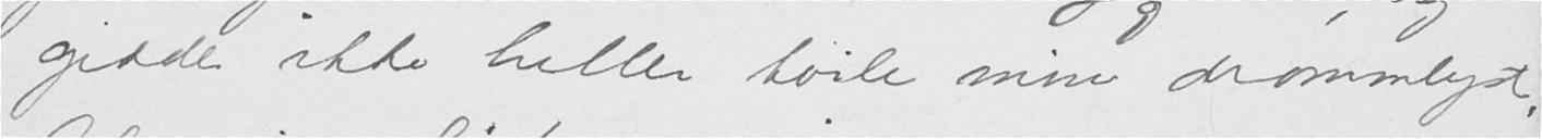gidder ikke heller tøile min drømmelyst.
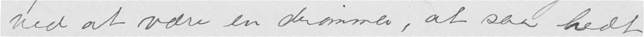ved at være en drømmer, at saa hedt
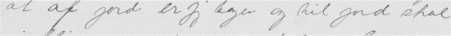at af jord er jeg tagen og til jord skal
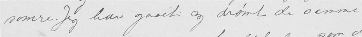somre. Jeg har gaaet og drømt de samme
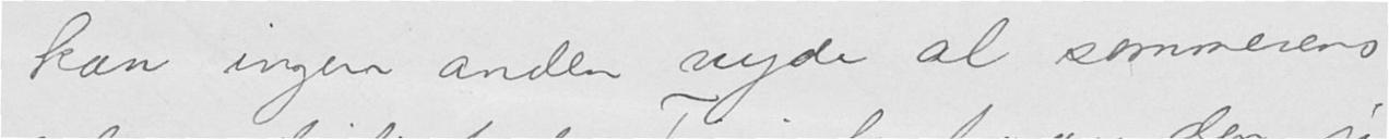kan ingen anden nyde al sommerens
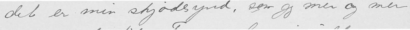det er min skjødesynd, som jeg mer og mer
